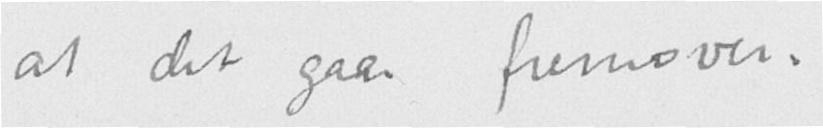at det gaar fremover.
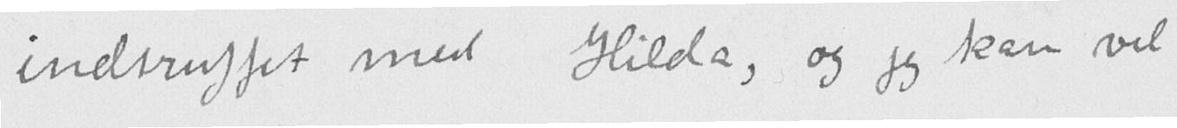indtruffet med Hilda, og jeg kan vel
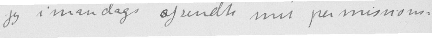jeg i mandags afsendte mit permissions-
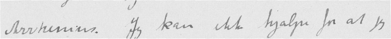Arrhenius. Jeg kan ikke hjælpe for at jeg
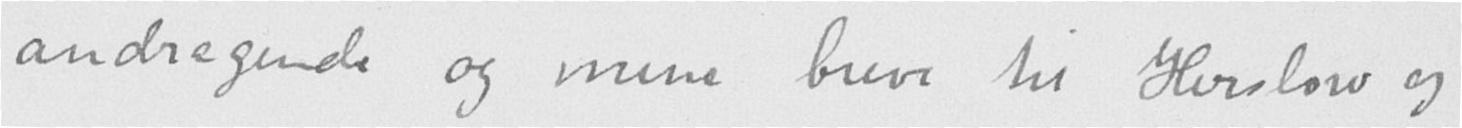andragende og mine breve til Herslow og
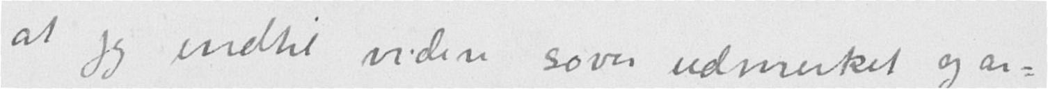at jeg indtil videre sover udmerket og ar-
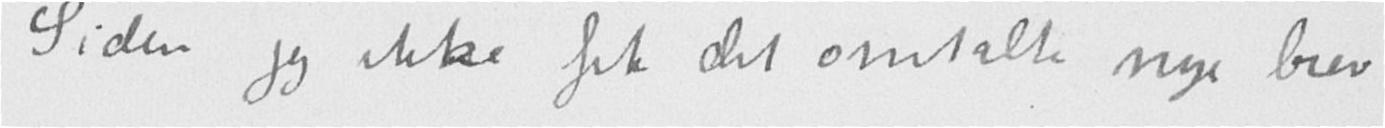Siden jeg ikke fik det omtalte nye brev
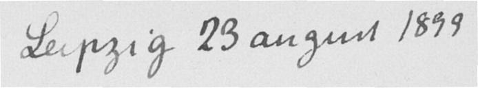Leipzig 23 august 1899
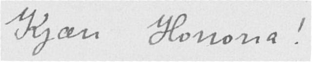Kjære Honoria!
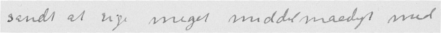sandt at sige meget middelmaadigt med
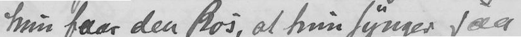hun faar den Ros, at hun synger saa
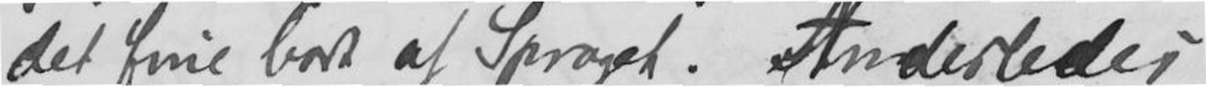det fine bort af Sproget. Anderledes
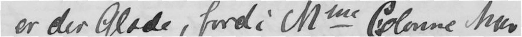er der Glæde, fordi Mme Colonne har
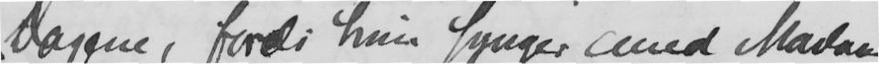Dagene, fordi hun synger med Madam
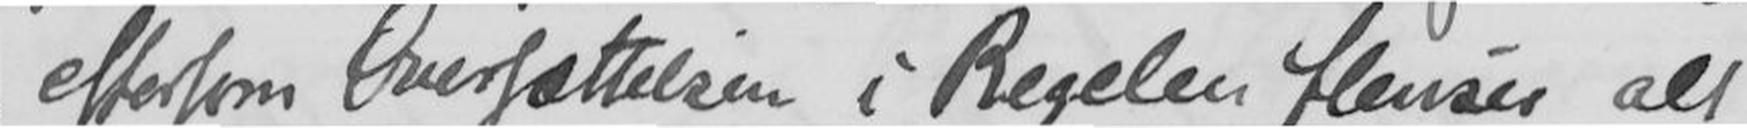eftersom Oversættelsen i Regelen flenser alt
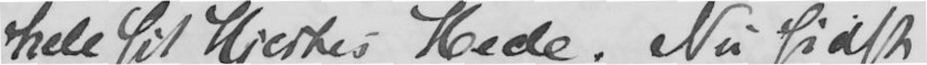hele sit Hjertes Hede. Nu sidst
